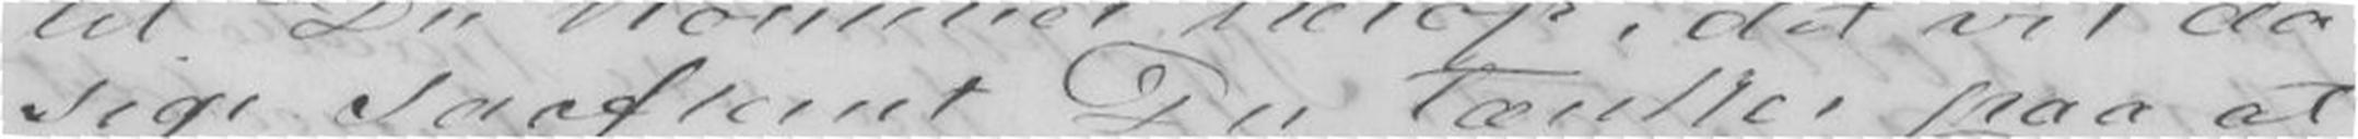sige saafremt Du tanker paa at
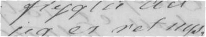jeg er ret nys-
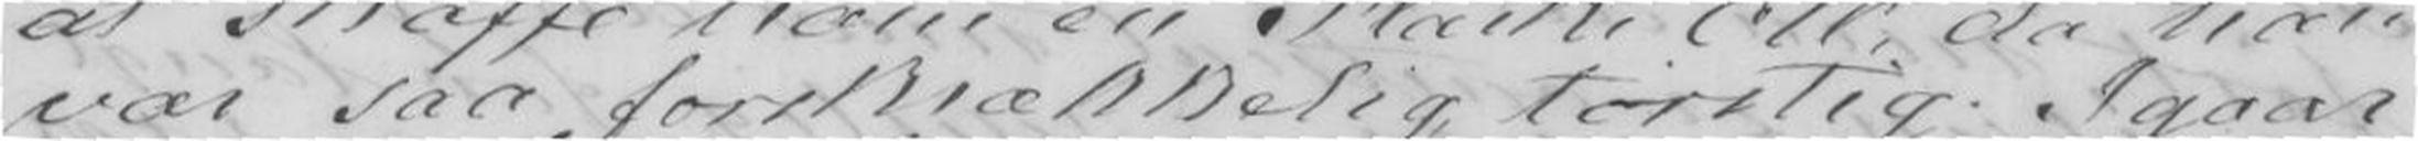var saa forskrækkelig tørstig. Igaar
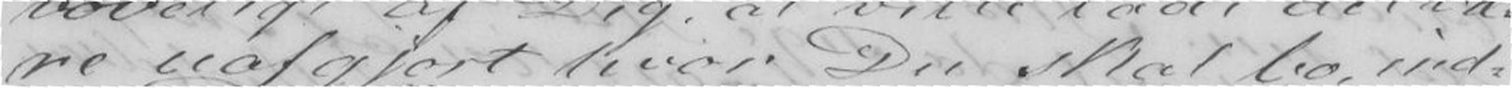re uafgjort hvor Du skal bo, ind-
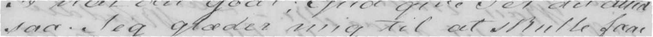saa. Jeg glæder mig til at skulle faae
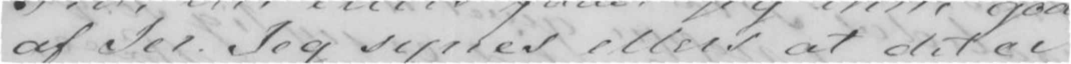af Jer. Jeg synes allers at det er
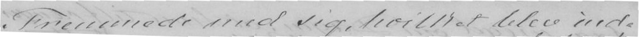Fremmede med sig, hvilket blev ind-
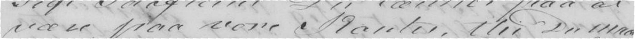være paa vore Kanter, thi Du maa
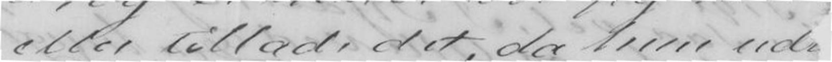eller tillade det, da hun udr
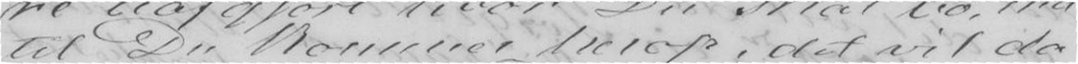til Du kommer herop, det vil da
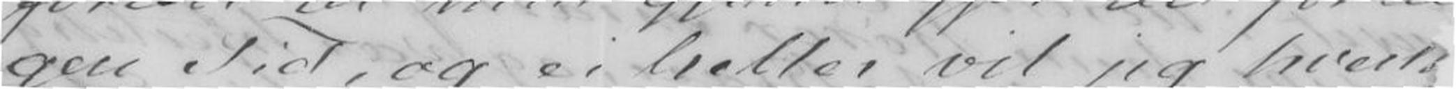gen Tid, og ei heller det, vil jeg hvert
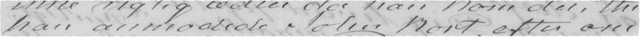han anmodede John kort efter om
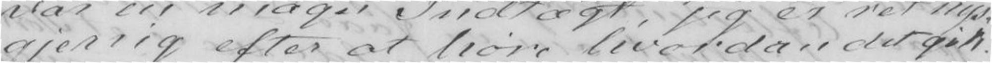gjerreig efter at høre hvordan det gik.
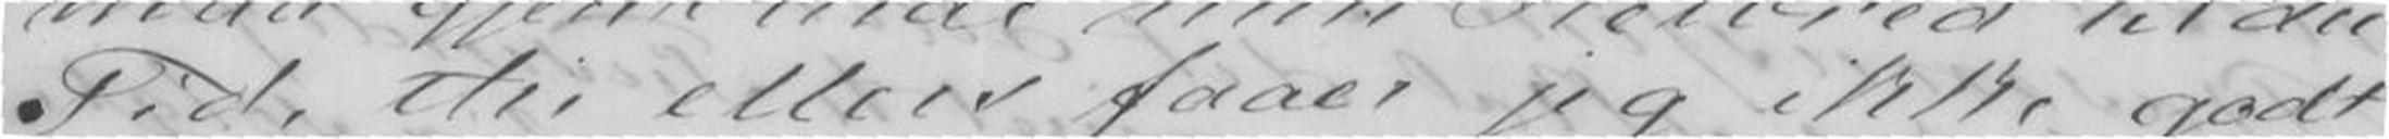Tid, thi ellers faaer jeg ikke godt
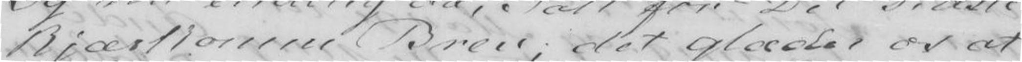kjærkomne Brev; det glæder os at
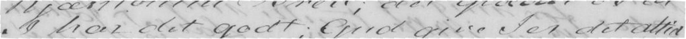I har det godt; Gud give Jer det altid
